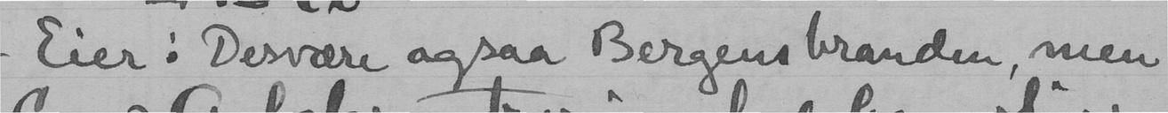Eier: Desvære ogsaa Bergens branden, men
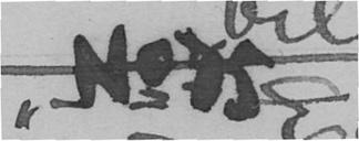No 75
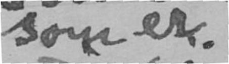som er
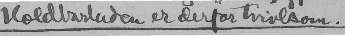Holdbarsheden er derfor tvivlsom.
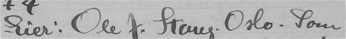Eier. Ole J. Stang. Oslo. Som
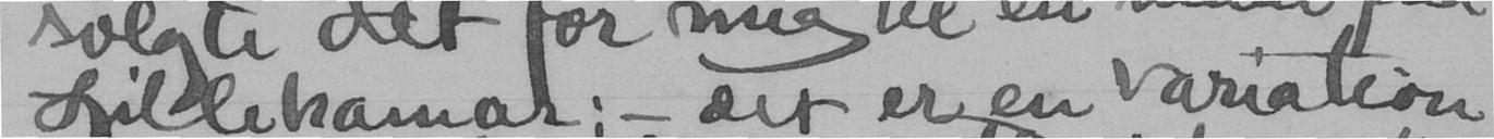Lillehamar; - det er en variation
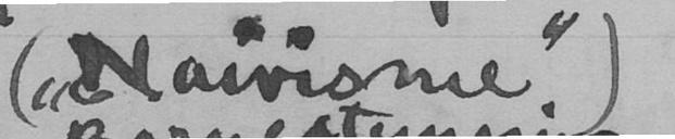("Naivisme".)
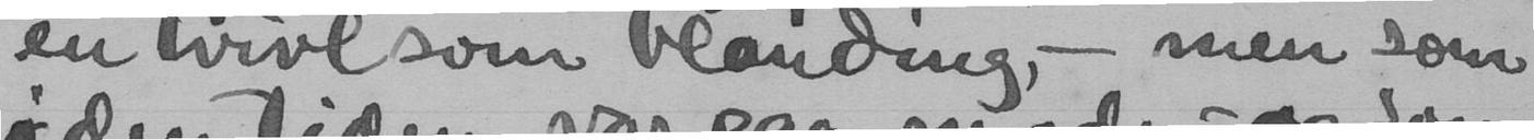en tvivlsom blanding, - men som
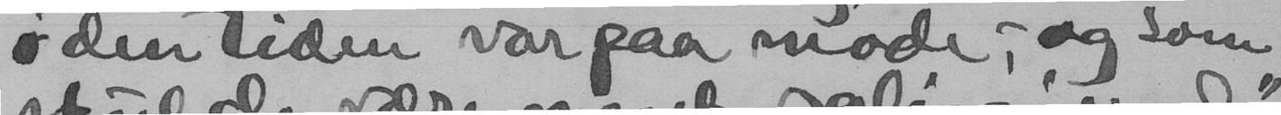i den tiden var paa mode, - og som
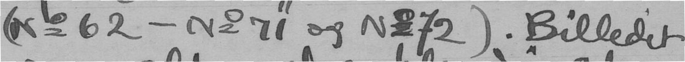(No 62 - No 71 og No 72). Billedet
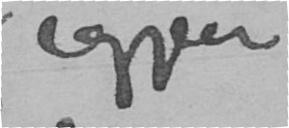igjen
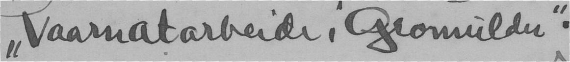"Vaarnatarbeide i Gromulden"
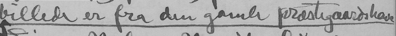billede er fra den gamle præstegaardshave
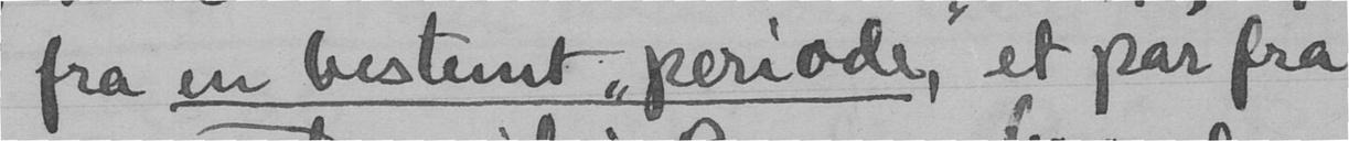fra en bestemt "periode" et par fra
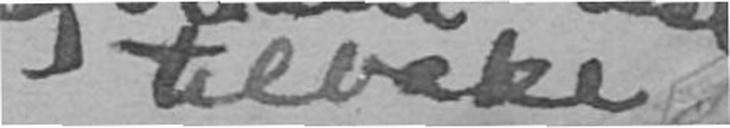tilbake
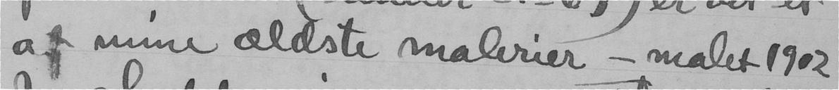af mine ældste malerier - malet 1902
